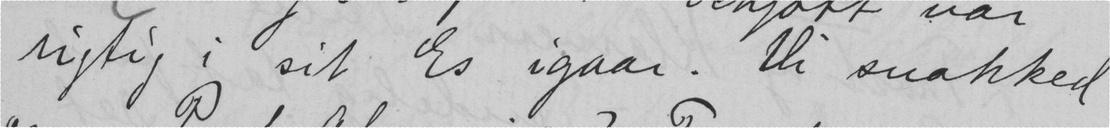rigtig i sit Es igaar. Vi snakked
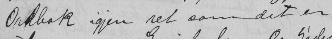Orkbok igjen ret som det er
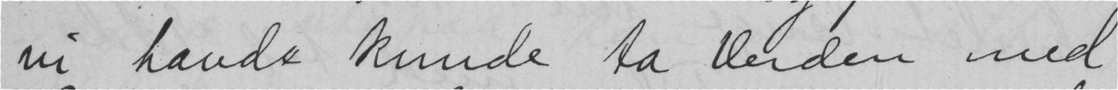vi havde kunde ta verden med
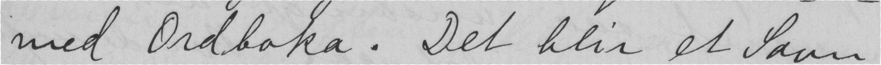med Ordboka. Det blir et Savn
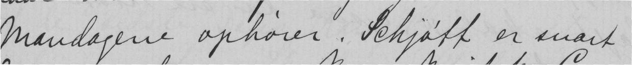mandagene ophøier. Schjøtt er snart
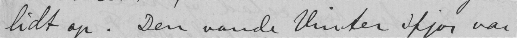lidt op. Den vonde vinter ifjor var
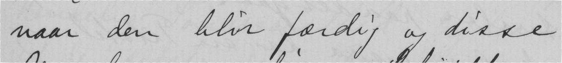naar den blir færdig og disse
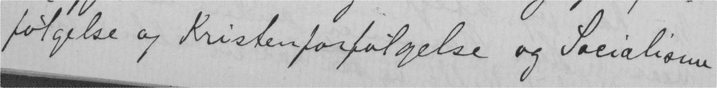følgelse og Kristenforfølgelse og Socialisme
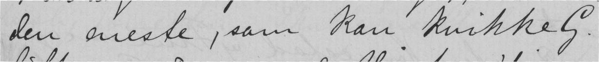den eneste, som kan kvikke G.
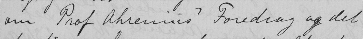om Prof Ahrenius' Foredrag og det
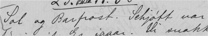Sol og Barfrost. Schjøtt var
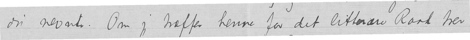du nevnte. Om jeg træffer henne før det litterære Raad trer
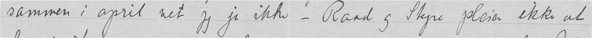sammen i april vet jeg jo ikke – Raad og Styre pleier ikke at
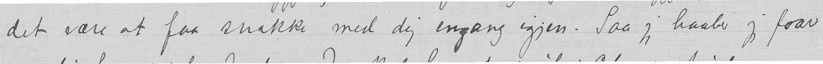det være at faa snakke med dig engang igjen. Saa jeg haaber jeg faar
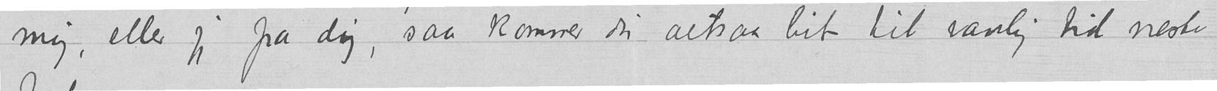mig, eller jeg fra dig, saa kommer du altsaa hit til vanlig tid neste
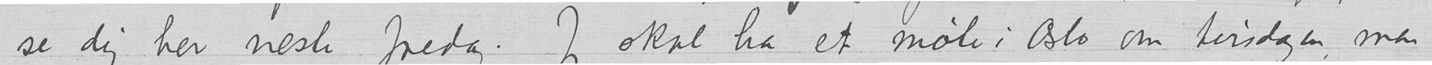se dig her neste fredag. Jeg skal ha et møle i Oslo om tirsdagen, men
